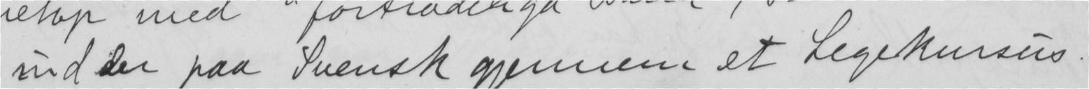ud der paa Svensk gjennem et Legekursus.
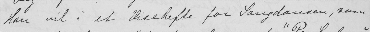Han vil i et Visehefte for Sangdansen, som
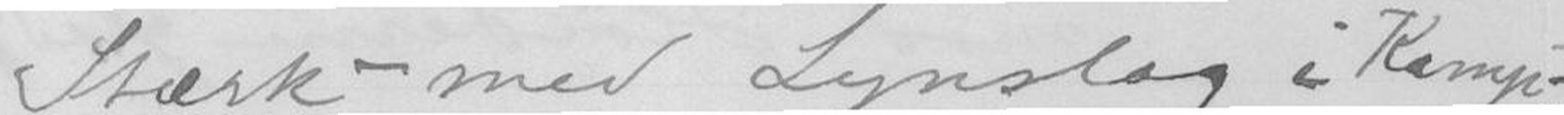Stærk - med Lynslag i Kamp -
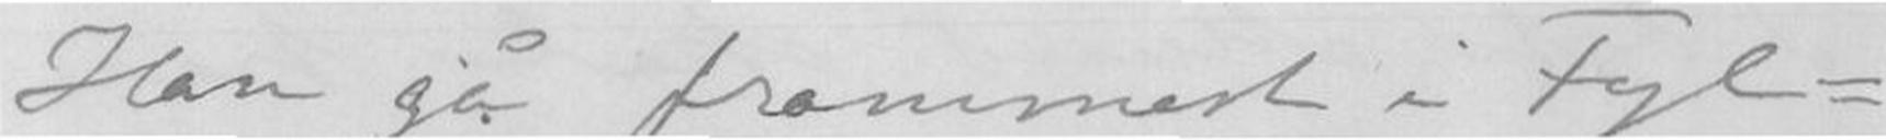Han gå frammest i Fyl-
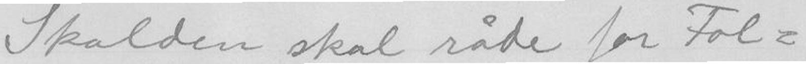Skalden skal råde for Fol-
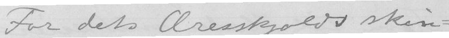For dets Æresskjolds Skin-
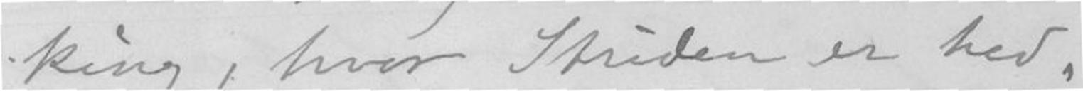king, hvor Striden er hed.
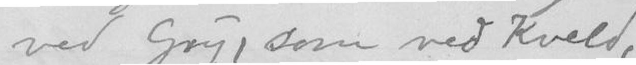ved Gry, som ved Kveld,
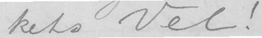kets Vel!
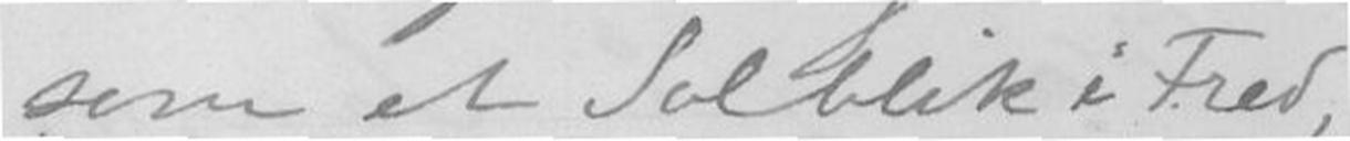som et Solblik i Fred,
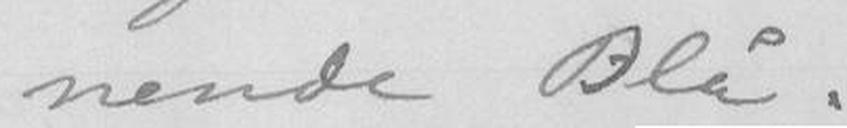nende Blå.
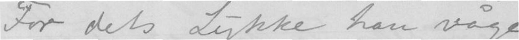For dets Lykke han våge
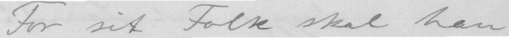For sit Folk skal han
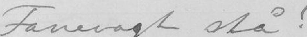Fanevagt stå!
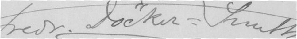Fredr. Döcker=Smith
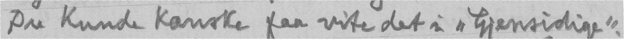Du kunde kanske faa vite det i "Gjensidige".
